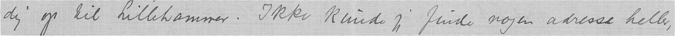dig op til Lillehammer. Ikke kunde jeg finde nogen adresse heller,
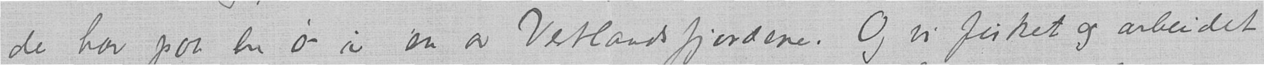de har paa en ø i en av Vestlandsfjordene. Og vi fisket og arbeidet
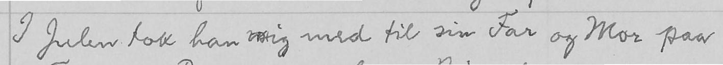I Julen tok han mig med til sin Far og Mor paa
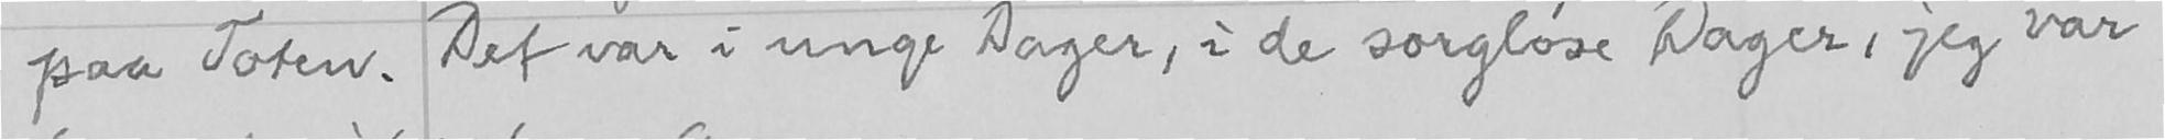paa Toten. Det var i unge Dager, i de sorgløse Dager, jeg var
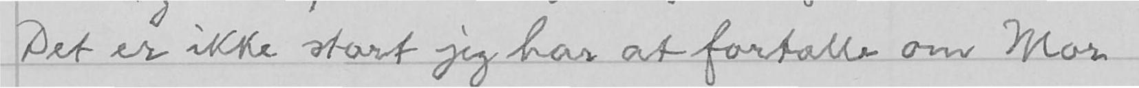Det er ikke stort jeg har at fortælle om Mor
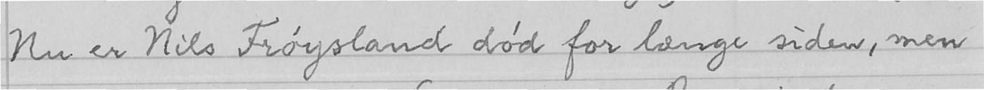Nu er Nils Frøysland død for længe siden, men
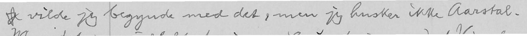j vilde jeg begynde med det, men jeg husker ikke Aarstal.
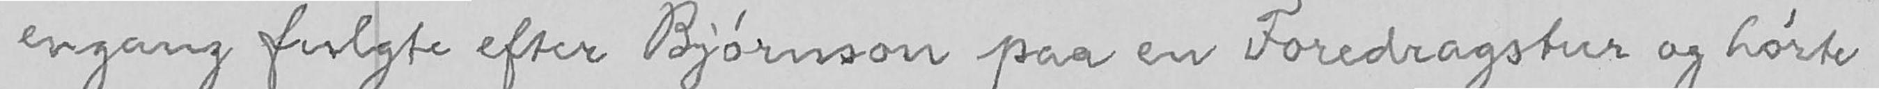engang fulgte efter Bjørnson paa en Foredragstur og hørte
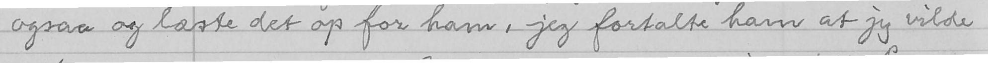ogsaa og læste det op for ham, jeg fortalte ham at jeg vilde
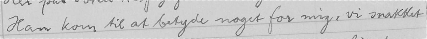Han kom til at betyde noget for mig, vi snakket
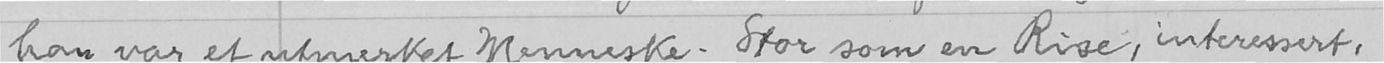han var et utmerket Menneske. Stor som en Rise, interessert,
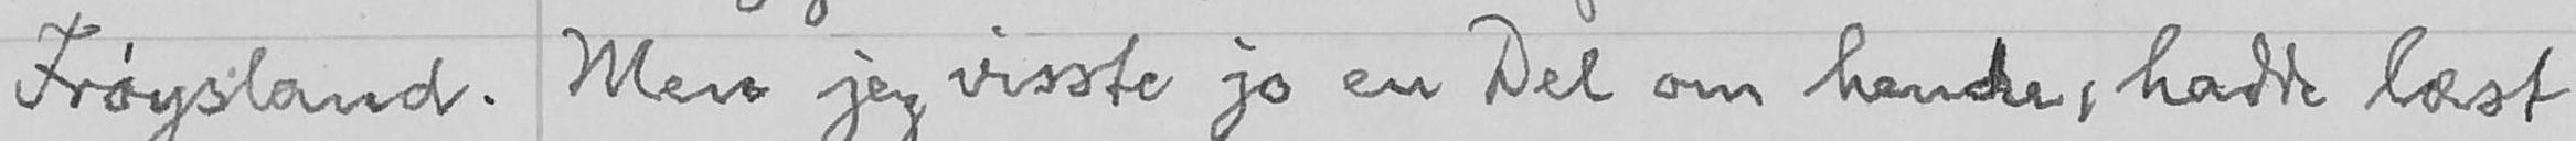Frøysland. Men jeg visste jo en Del om hende, hadde læst
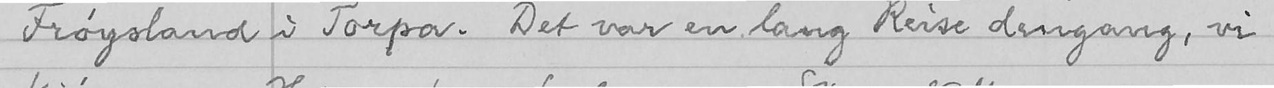Frøysland i Torpa. Det var en lang Reise dengang, vi
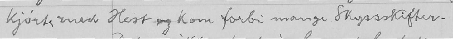kjørte med Hest og kom forbi mange Skyssskifter.
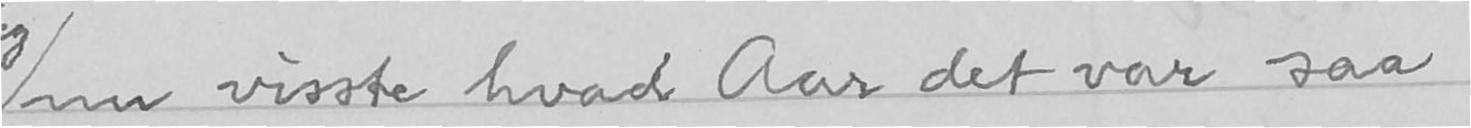nu visste hvad Aar det var saa
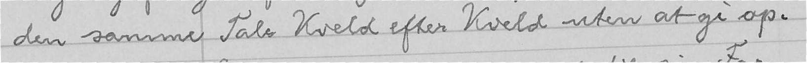den samme Tale Kveld efter Kveld uten at gi op.
